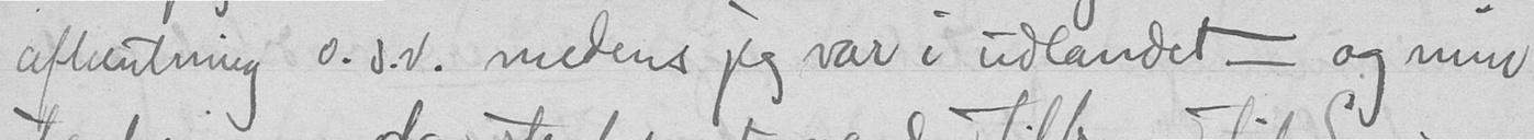afhentning o.s.v. medens jeg var i udlandet og min
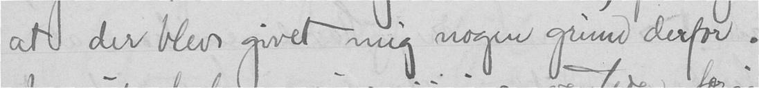at der blev givet mig nogen grune derfor.
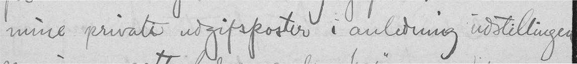mine private udgifsposter i anledning udstillinger
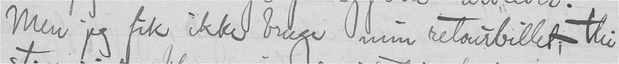Men jeg fik ikke bruge min retourbillet; thi
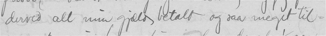derved all min gjæld betalt og saa meget til-
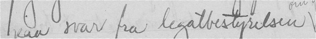paa svar fra legatbestyrelsen
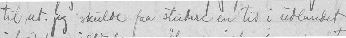til, at jeg skulde faa studere en tid i udlandet
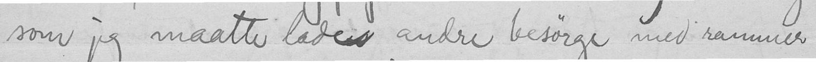som jeg maatte lades andre besørge med sommer
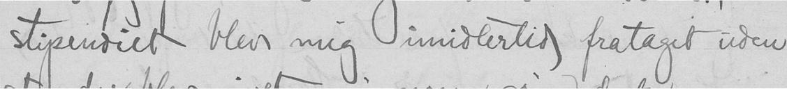stipendiet blev mig imidlertid frataget uden
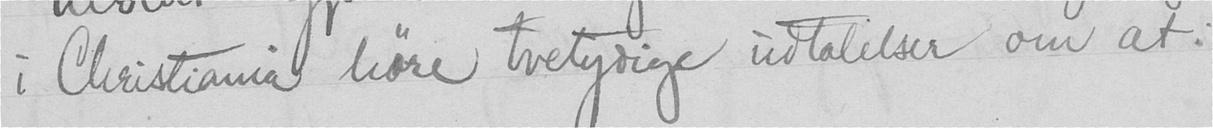i Christiania høre tvetydige udtalelser om at:
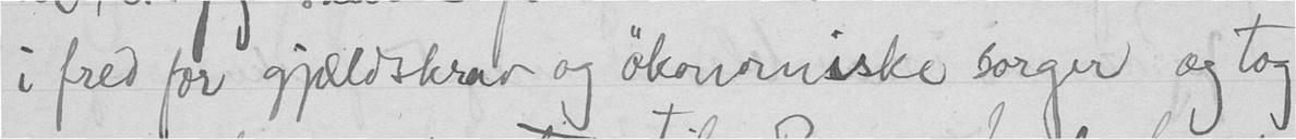i fred for gjældskrav og økonomiske sorger og tog
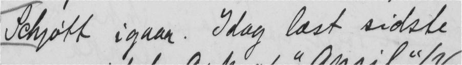Schjøtt igaar. Idag læst sidste
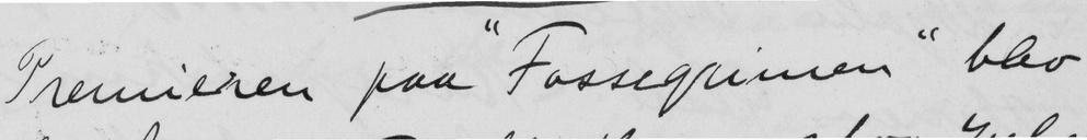Premieren paa "Fossegrimen" blev
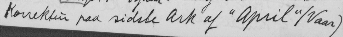Korrektur paa sidste Ark af "April" (Vaar)
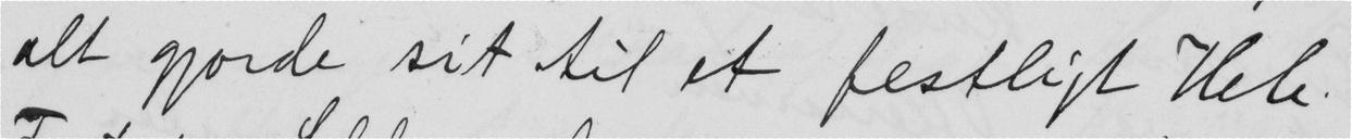alt gjorde sit til et festligt Hele.
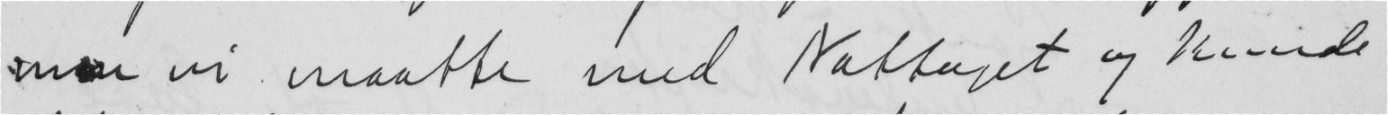men vi maatte med Nattoget og kunde
